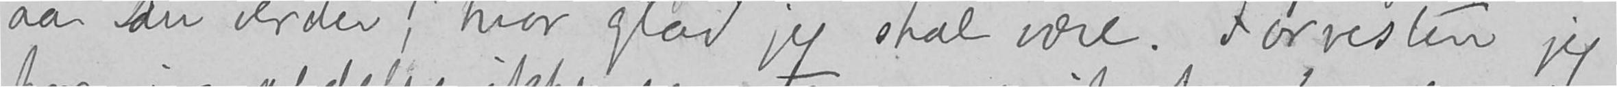aa du verden, hvor glad jeg skal være. Forresten jeg
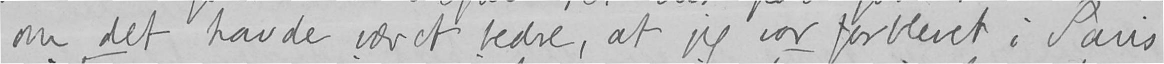om det havde været bedre, at jeg var forblevet i Paris
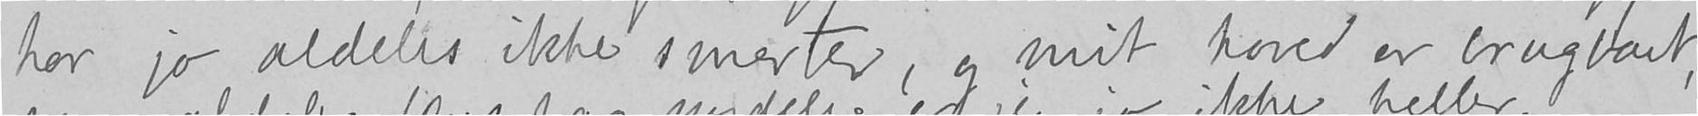har jo aldeles ikke smerter, og mit hoved er brugbart,
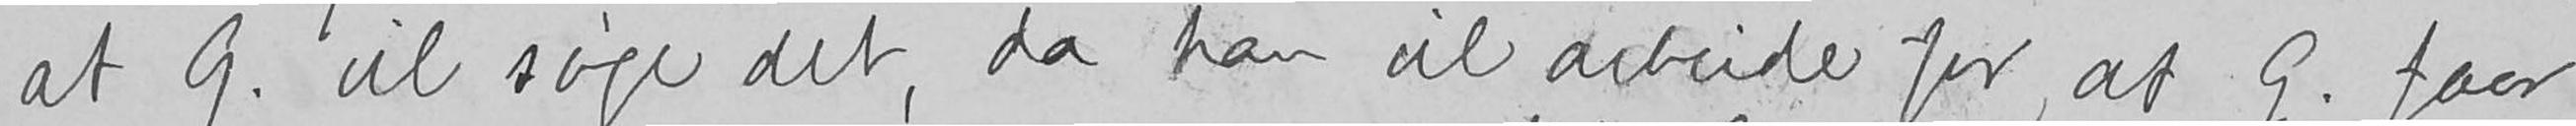at G. vil søge det, da han vil arbeide for, at G. faar
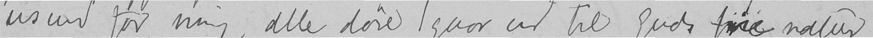usund for mig, alle døre gaar ud til Guds frie natur,
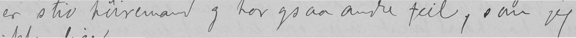er stiv høiremand og har ogsaa andre feil, som jeg
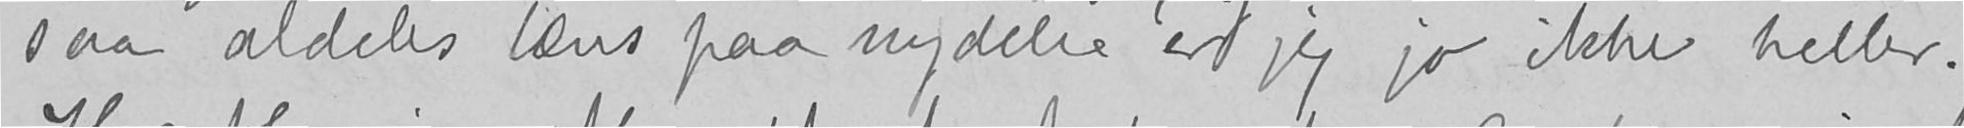saa aldeles læns paa nydelse er jeg jo ikke heller.
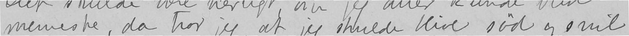menneske, da tror jeg, at jeg skulde blive sød og snil,
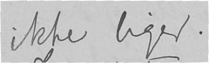ikke liger.
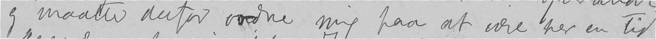og maatte derfor ordne mig paa at være her en tid.
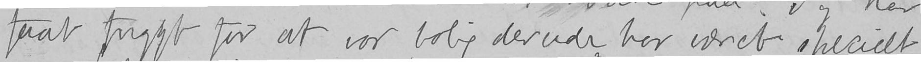faat frygt for at vor bolig derude har været specielt
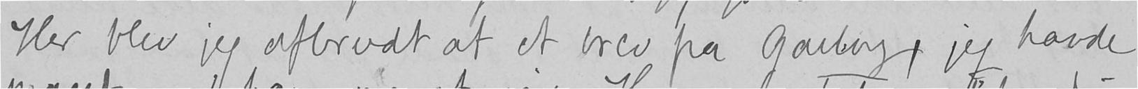Her blev jeg afbrudt af et brev fra Garborg, jeg havde
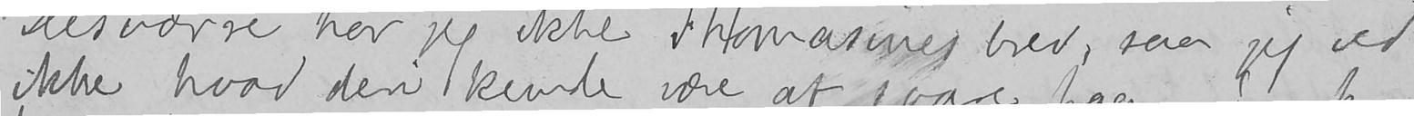Desværre har jeg ikke Thomasines brev, saa jeg ved
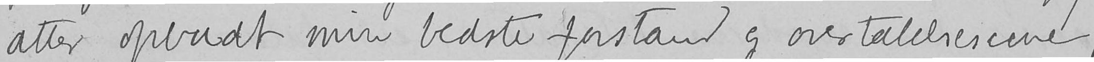atter opbudt min bedste forstand og overtalelsesevne,
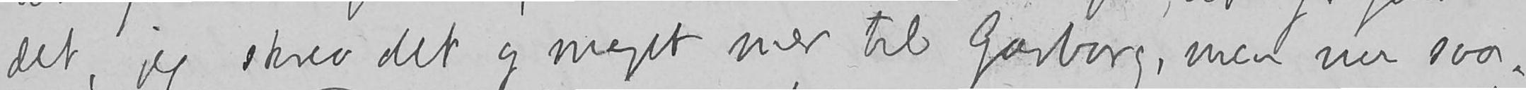det, jeg skrev det og meget mer til Garborg, men nu sva-
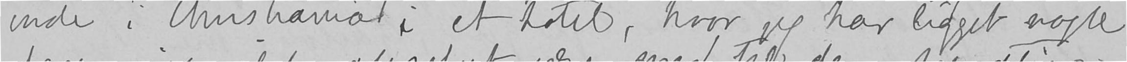inde i Christiania i et hotel, hvor jeg har ligget nogle
